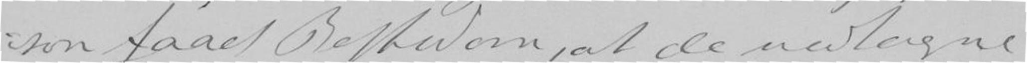=son faaet Besked om, at de mottagne
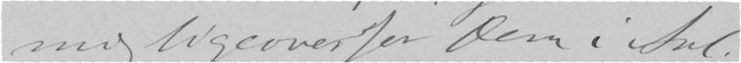mig ligeoverfor Dem i Anl
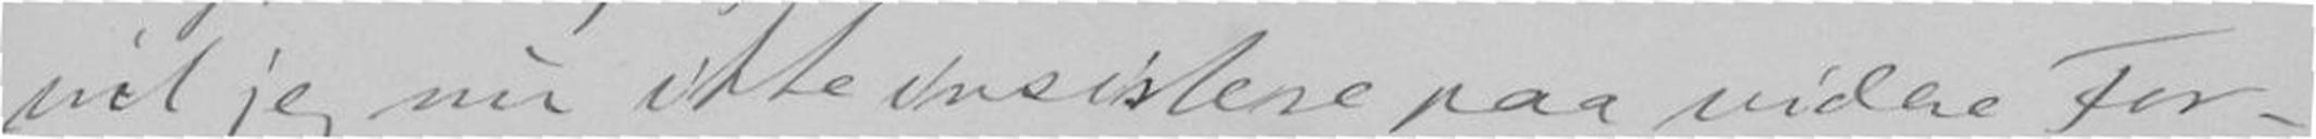vil jeg nu ikke insistere paa videre For-
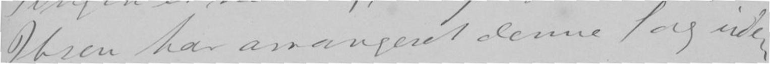Ibsen har arrangeret denne I og uden
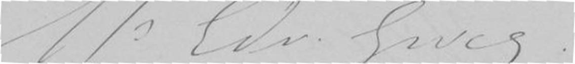Mr Edv. Grieg !
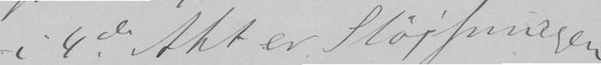i 4de Akt er Sløyfningen
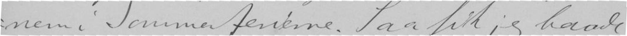=nem i Sommerferierne. Saa fik jeg baade
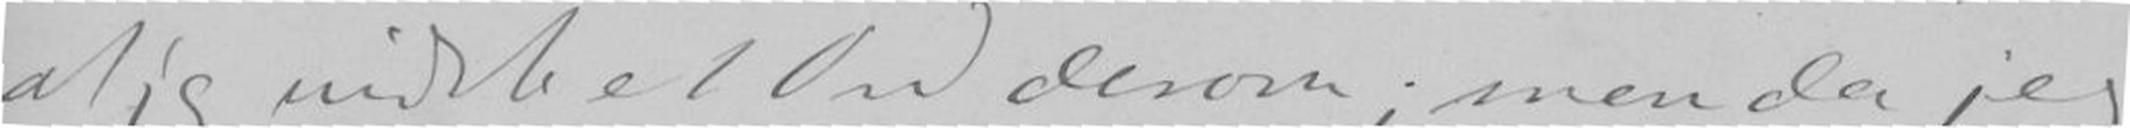at jeg vidste et Ord derom; men da jeg
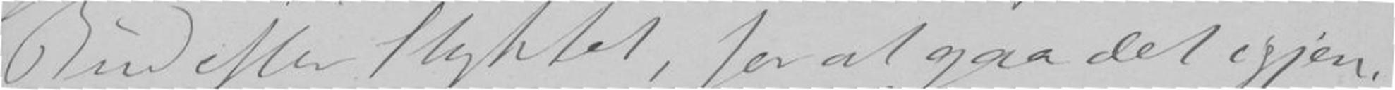Bud efter Stykket, for at gaa det igjen-
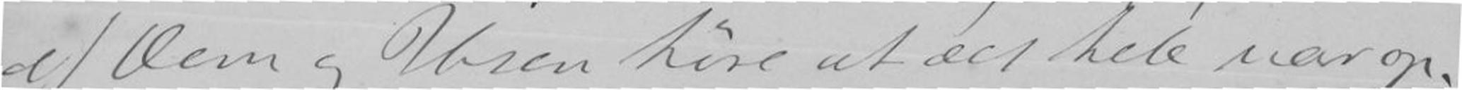af Dem og Ibsen høre at det hele var op-
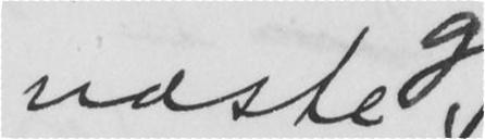næste
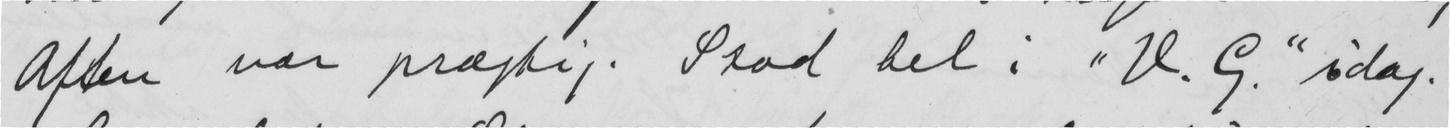Aften var prægtig. Stod hel i "V. G. idag.
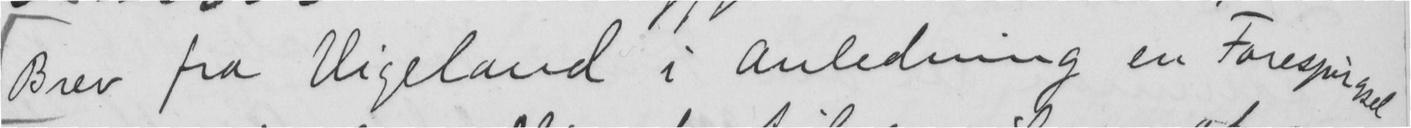Brev fra Vigeland i Anledning en Forespørgsel
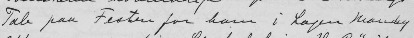Tale paa Festen for ham i Lagen Mandag
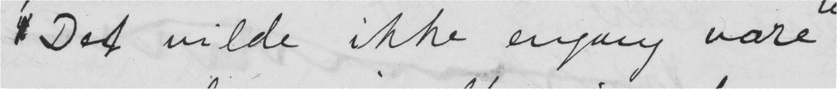Det vilde ikke engang være
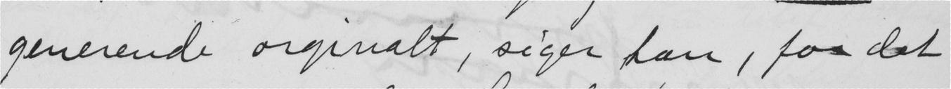generende orginalt, siger han, for det
